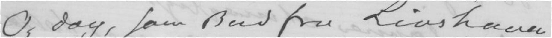Og dog, som Bud fra Livshaven
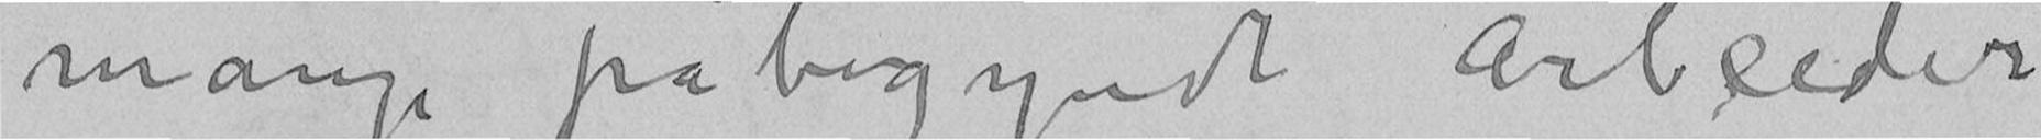mange påbegyndte Arbeider
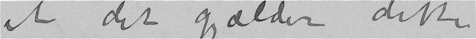at det gjælder dette
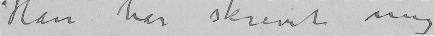Han har skrevet mig
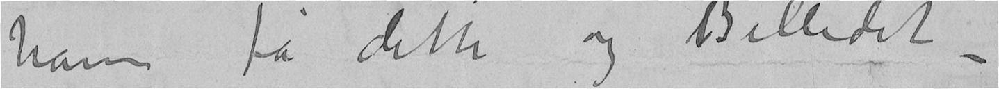ham få dette og Billedet –
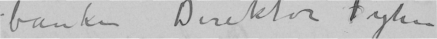banken Direktør Fyhn
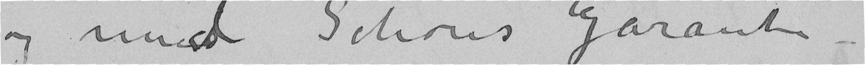og mod Schous Garanti –
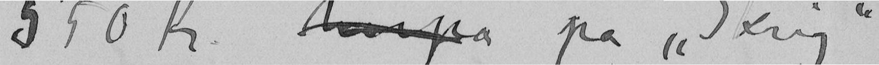550 Kr. herpå på «Skrig»
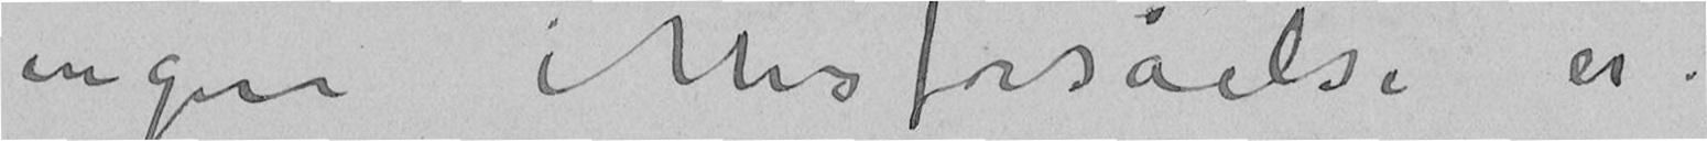ingen Misforståelse er.
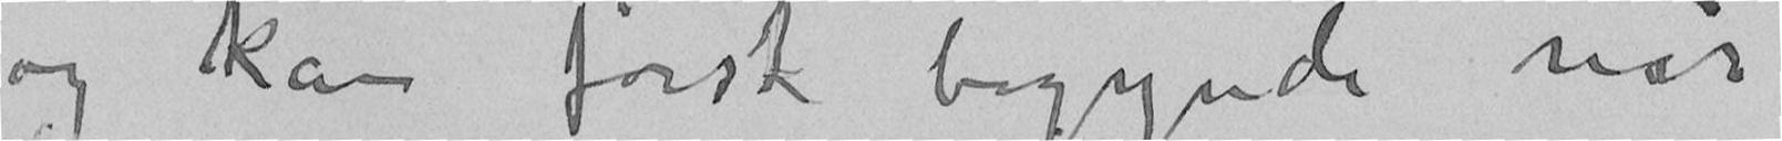og kan først begynde når
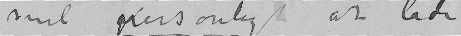snil personligt at lade
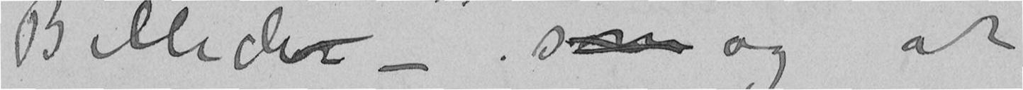Billeder – som og at
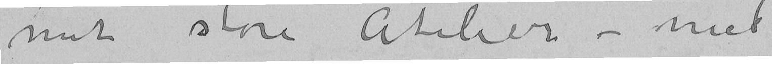mit store Atelier – med
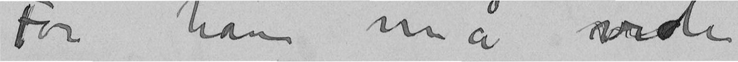For han må vide
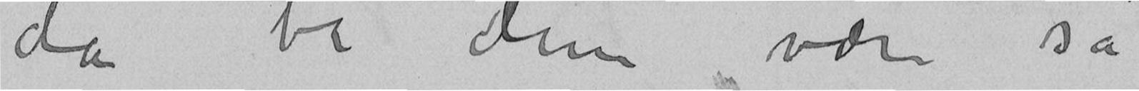da be Dem være så
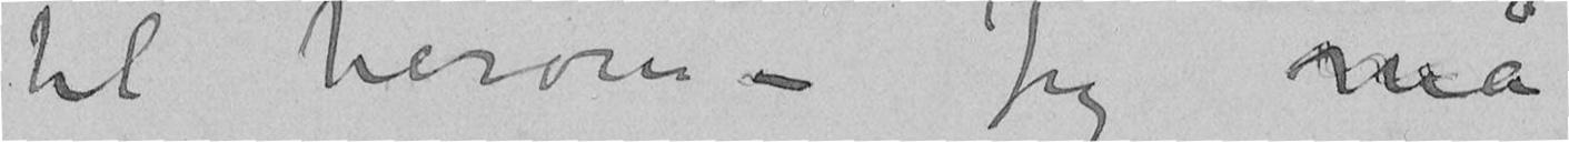til herom – Jeg må
